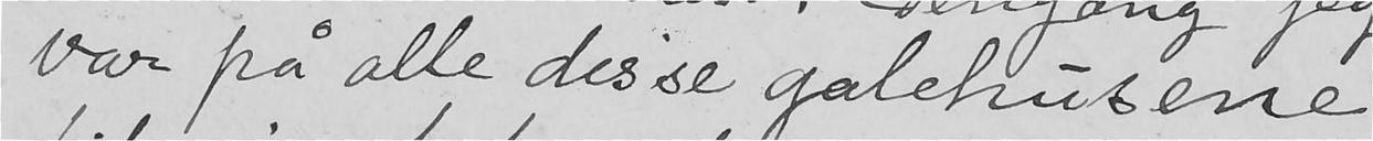var på alle disse galehusene
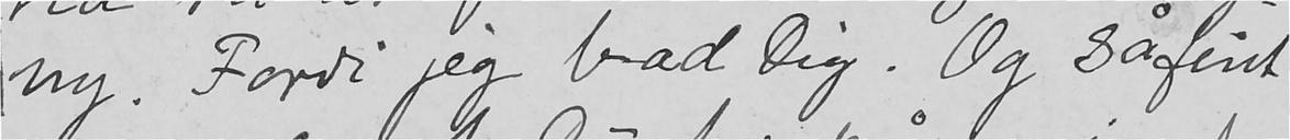ny. Fordi jeg bad Dig. Og så fint
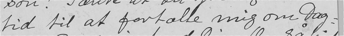tid til at fortælle mig om Dag-
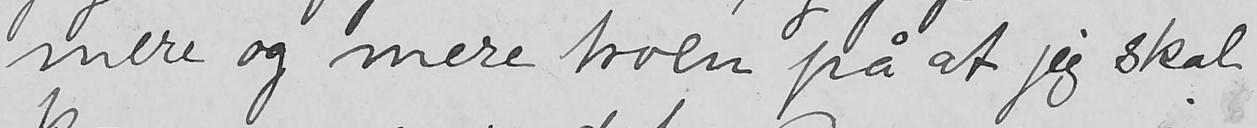mere og mere troen på at jeg skal
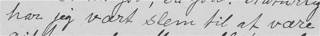har jeg vært slem til af være
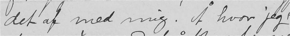det af med mig. Å hvor jeg
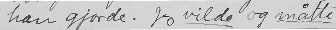han gjorde. Jeg vilde og måtte
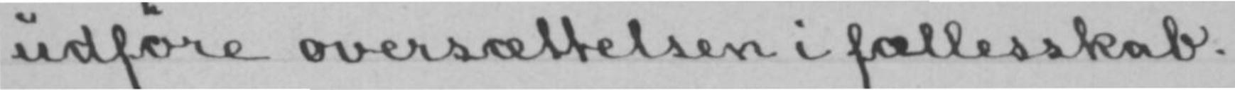udføre oversættelsen i fællesskab.
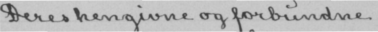Deres hengivne og forbundne
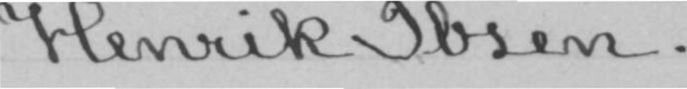Henrik Ibsen.
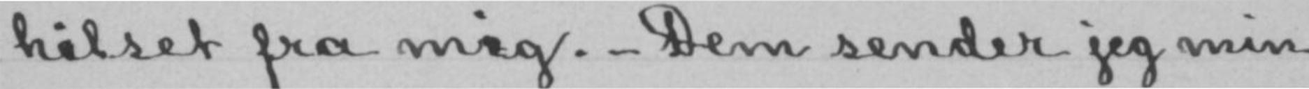hilset fra mig.- Dem sender jeg min
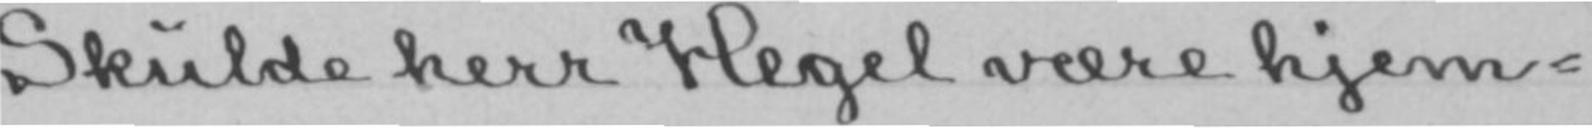Skulde herr Hegel være hjem-
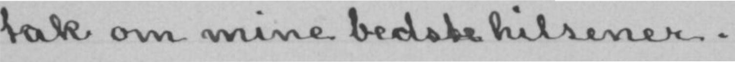tak om mine bedste hilsener.
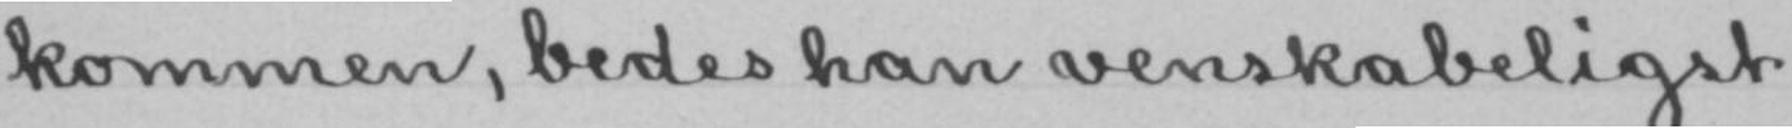kommen, bedes han venskabeligst
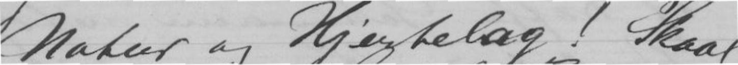Natur og Hjertelag! Skaal
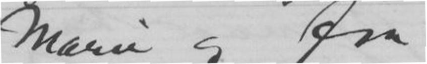Marie og fra
